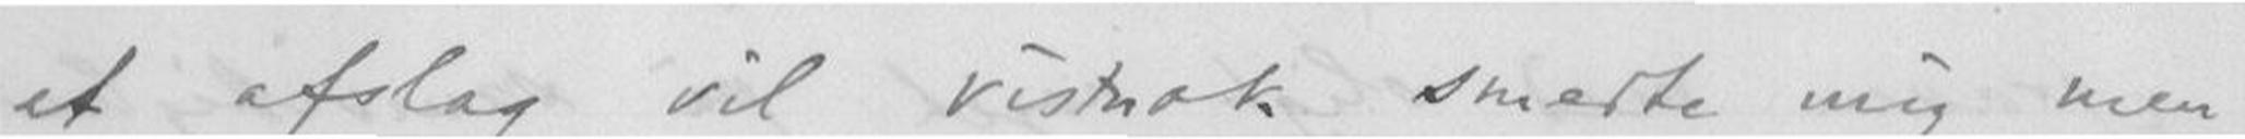et afslag vil vistnok smerte mig men
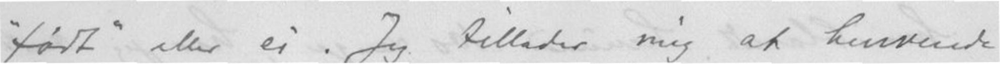"født" eller ei. Jeg tillader mig at henvende
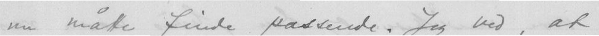nu måtte finde passende. Jeg ved, at
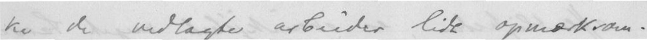de vedlagte arbeider lidt opmærksom-
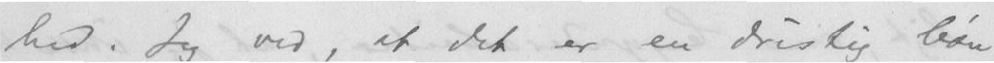hed. Jeg ved, at det er en dristig bøn
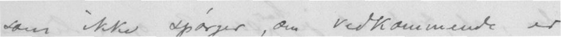som ikke spørger, om vedkommende er
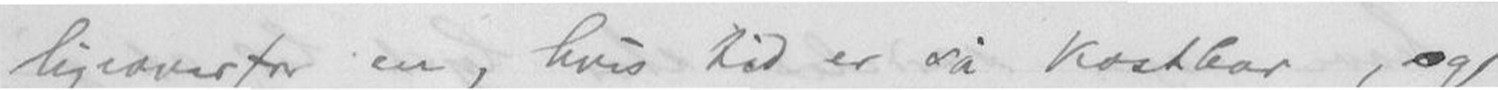ligeoverfor en, hvis tid er så kostbar, og
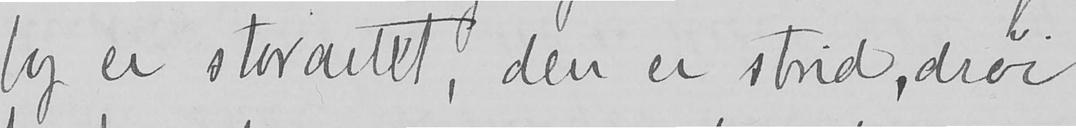bog er storartet, den er strid, drøi
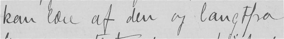kan lære af den og langtfra
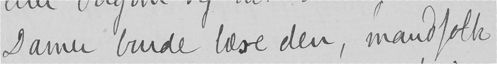Damer burde læse den, mandfolk
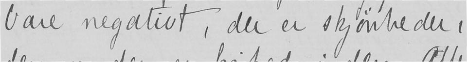bare negativt, der er skjønheder i
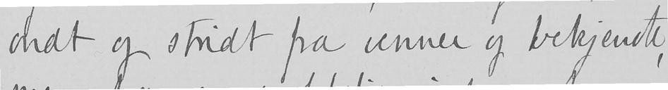ondt og stridt fra venner og bekjendte,
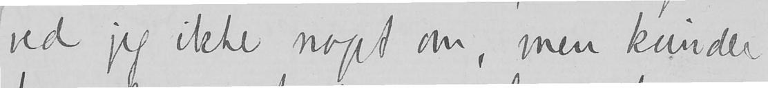ved jeg ikke noget om, men kvinder
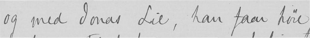og med Jonas Lie, han faar høre
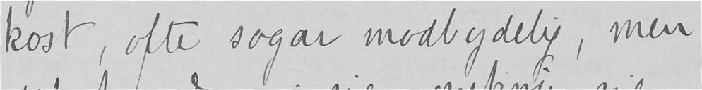kost, ofte sogar modbydelig, men
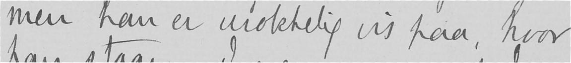men han er urokkelig vis paa, hvor
